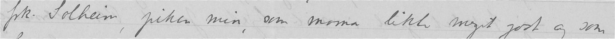frk. Solheim, piken min, som mama likte meget godt og som
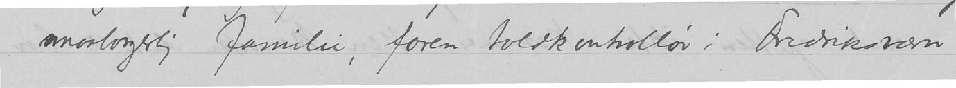smaaborgerlig familie, faren toldkonhollor i Fredriksværn
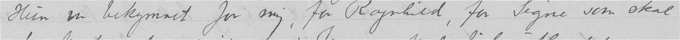Hun var bekymret for mig, for Ragnhild, for Signe som skal
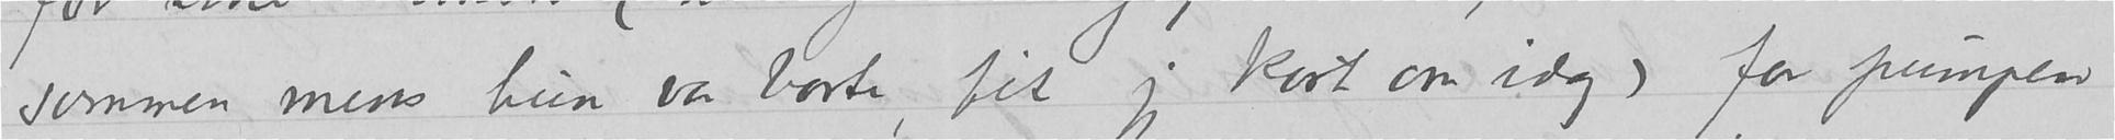sammen mens hun var borte, fik jeg kort om idag) for pumpen
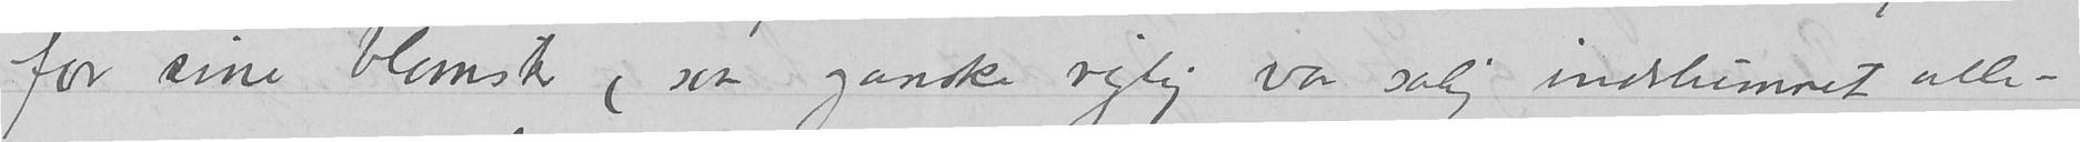for sine blomster (saa ganske rigtig var salig indslumret alle –
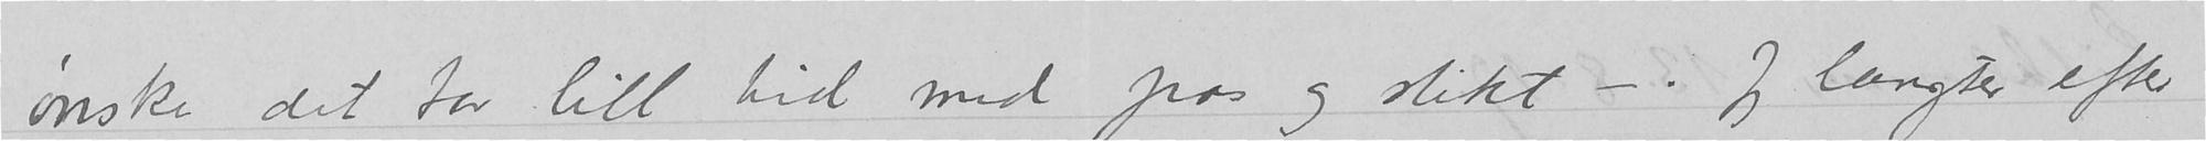ønske, det tar litt tid med pas og slikt –! Jeg længter efter
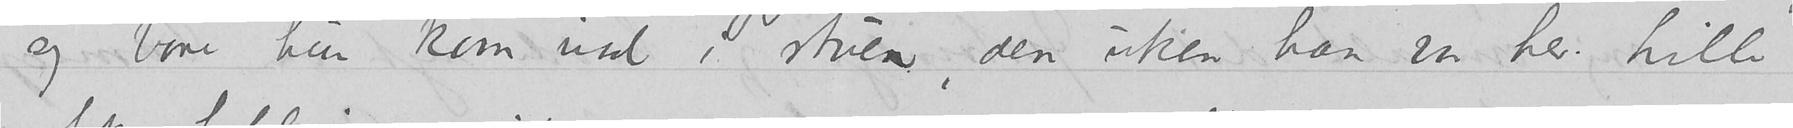og bare hun kom ind i stuen, den uken han var her Lille
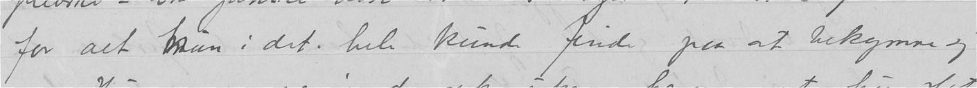for alt kun i det hele kunde pinde paa at bekymre sig
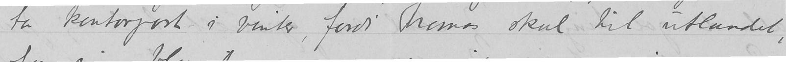for kontorpost i vinter, fordi Thomas skal til utlandet,
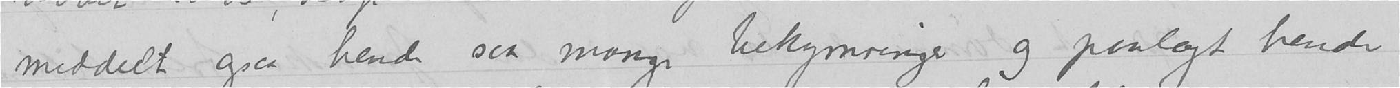meddelt ogsaa hende saa mange bekymringer og paalagt hende
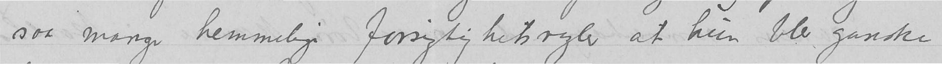saa mange hemmelige forsigtighetsregler at hun blev ganske
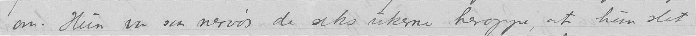om. Hun var saa nervøs de seks ukerne heroppe, at hun slet
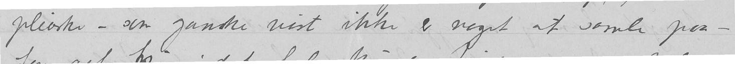pleirske – som ganske vist ikke er noget at samle paa –
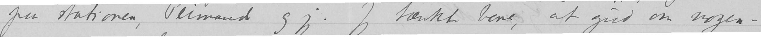paa stationen, Peimand og jeg. Jeg tænkte bare, at gud om nogen-
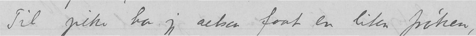Til pike har jeg altsaa faat en liten frøken,
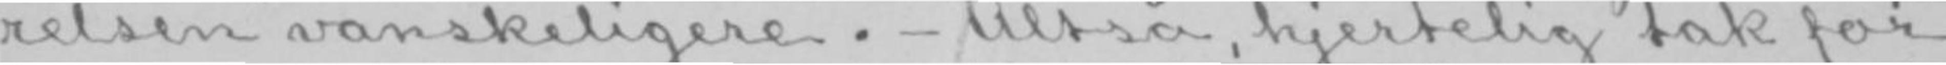relsen vanskeligere.- Altså, hjertelig tak for
